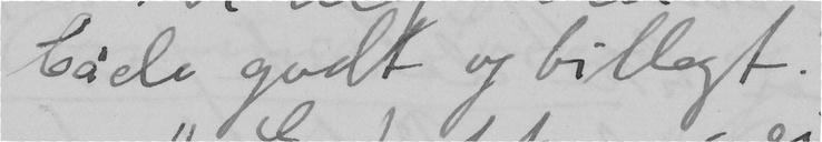både godt og billegt.
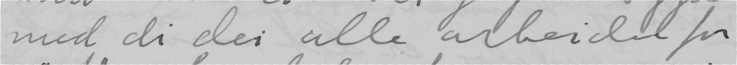med di dei alle arbeide for
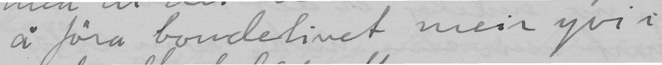å föra bondelivet meir yvi i
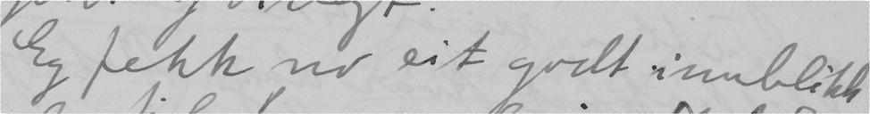Eg fekk no eit godt innblikk
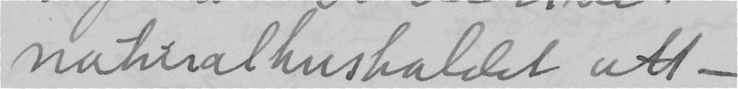naturalhushaldet att –
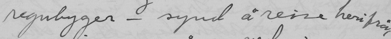regnbyger – synd å reise heimifrå,
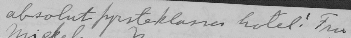absolut fyrsteklasses hotel! Fru
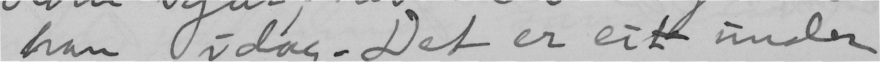han idag. Det er eitt under
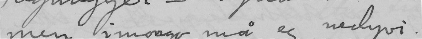men imorgo må eg nedyvi.
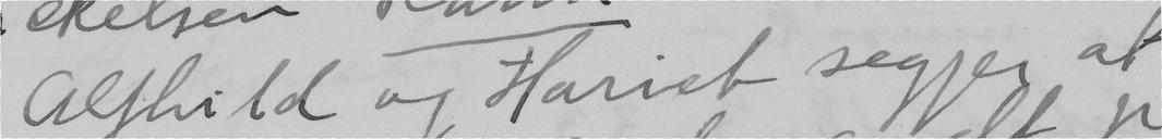Alfhild og Harriet segjer at
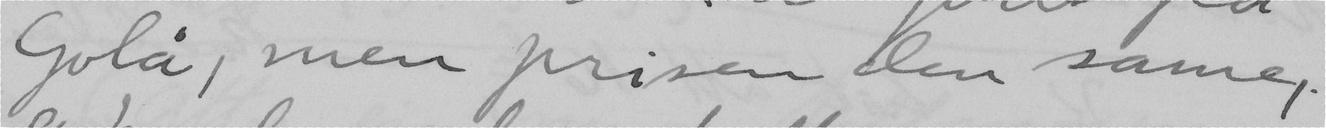Golå, men prisen den same,
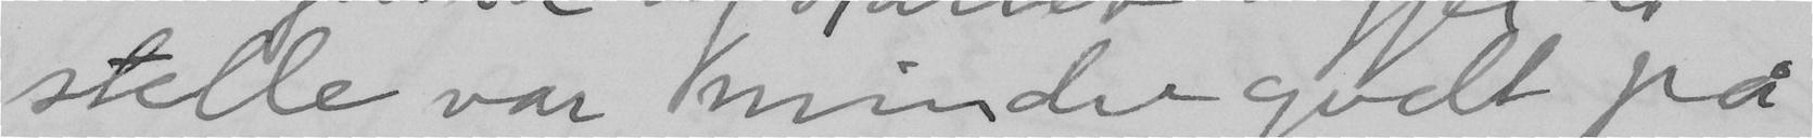stelle var mindre godt på
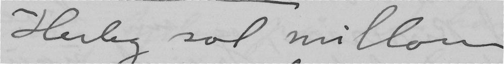Herleg sol millom
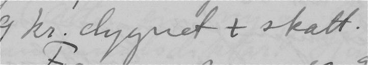9 kr. dygnet + skatt.
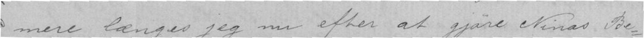mere længes jeg nu efter at gjøre Ninas Be}
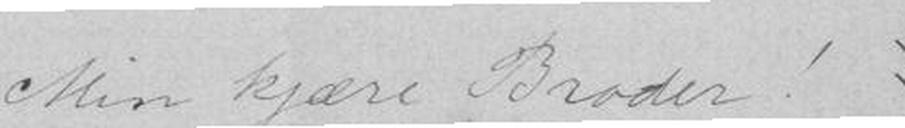Min kjære Broder!
